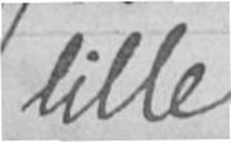lille
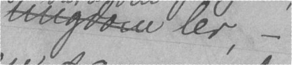ungdom ler, -
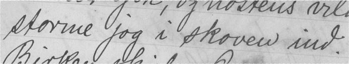storme jog i skoven ind.
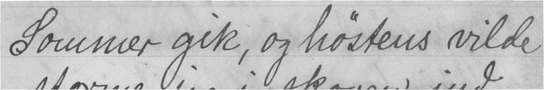Sommer gik, og høstens vilde
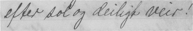efter sol og deligt veir!
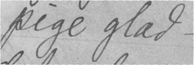pige glad -
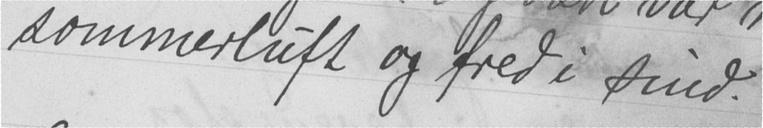sommerluft og fred i sind.
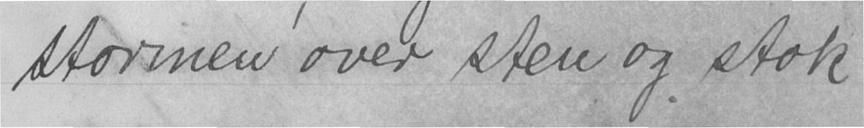stormen over sten og stok
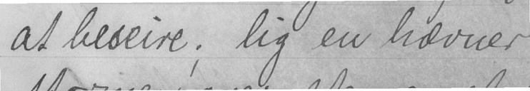at beseire; lig en hævner
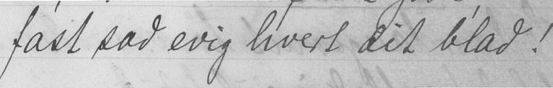fast sad evig hvert dit blad!
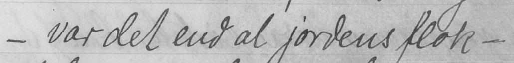- var det end al jordens flok -
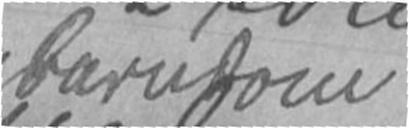barndom
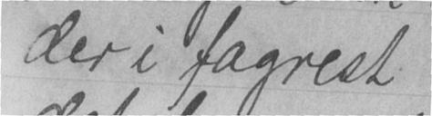der i fagrest
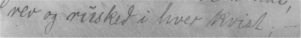rev og rusked i hver kvist; -
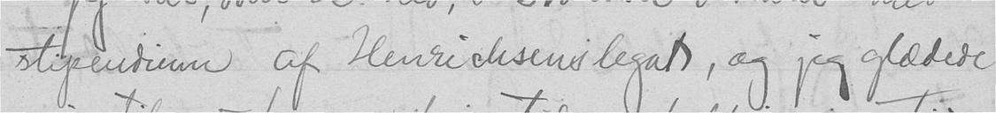stipendium af Henrichsenslegat, og jeg glædede
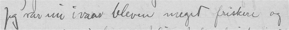Jeg var nu i vaar bleven meget friskere og
